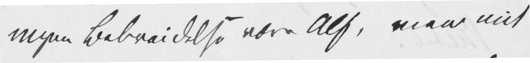ingen Bebreidelse være Alf, men mit
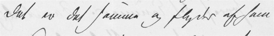Det er det samme og flyder af sam-
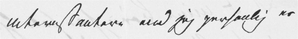interessantere end jeg personlig er
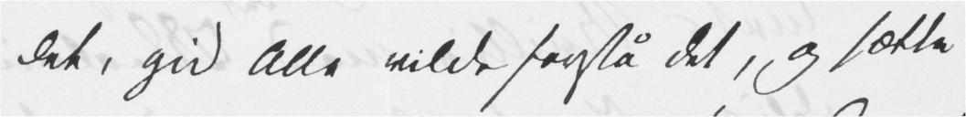det, gid Alle vilde forstå det, og sætte
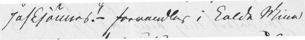paskjønnes! – forvandles i kolde Miner
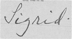Sigrid.
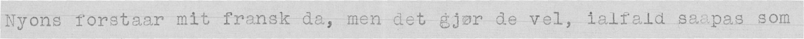Nyons forstaar mit fransk da, men det gjør de vel, ialfald saapas som
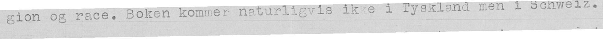gion og race. Boken kommer naturligvis ikke i Tyskland men i Schweiz.
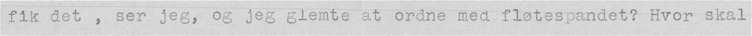fik det, ser jeg, og jeg glemte at ordne med fløtespandet? Hvor skal
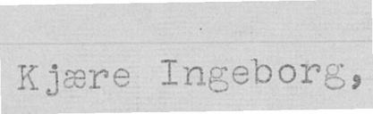Kjære Ingeborg,
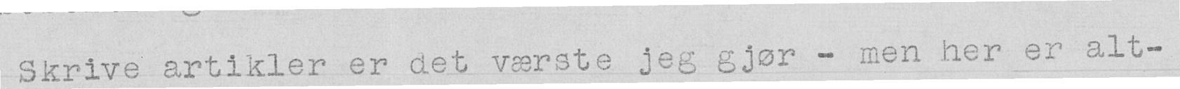Skrive artikler er det værste jeg gjør - men her er alt-
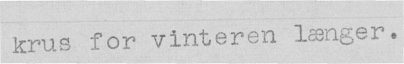krus for vinteren længer.
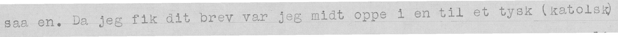saa en. Da jeg fik dit brev var jeg midt oppe i en til et tysk (katolsk)
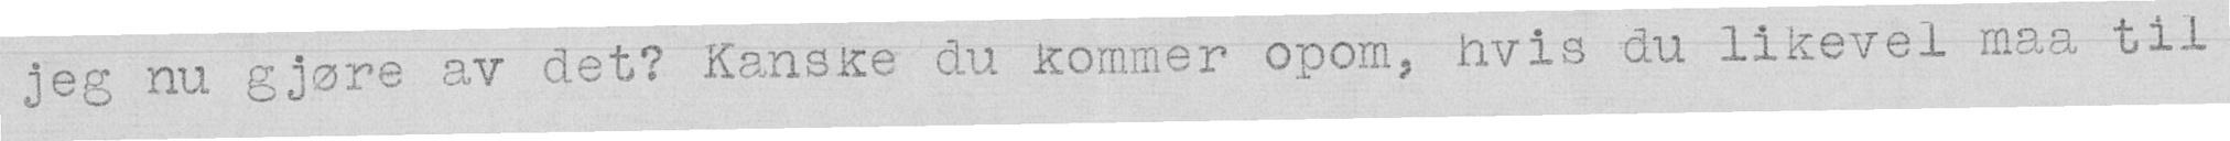jeg nu gjøre av det? Kanske du kommer opom, hvis du likevel maa til
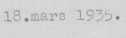18.mars 1935.
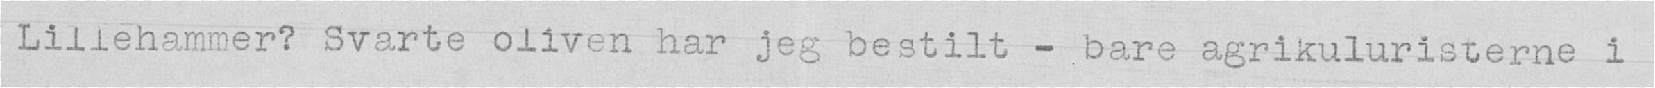Lillehammer? Svarte oliven har jeg bestilt - bare agrikuluristerne i
